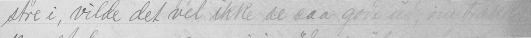stre i, vilde det vel ikke se saa godt ud, om trækket
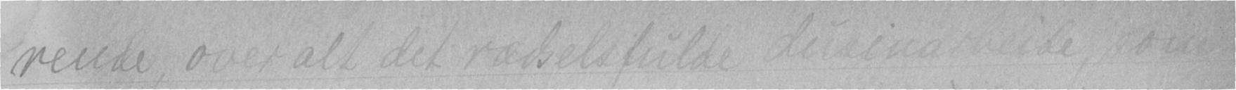rende over alt det rædselsfulde dusinarbeide,
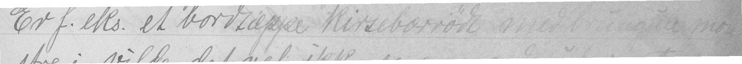Er f.eks. et bordtæppe kirsebærrødt med brungule møn-
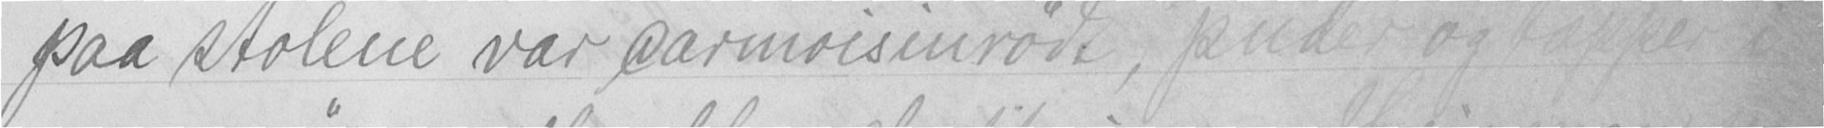paa stolene var carmoisinrødt, puder og tæpper i
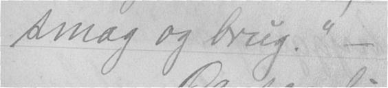smag og brug." -
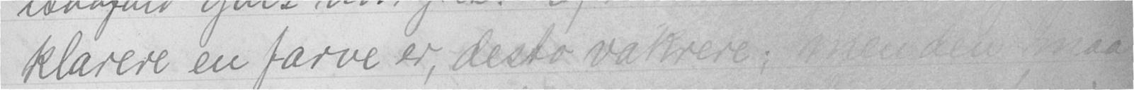klarere en farve er, desto vakrere; men den maa
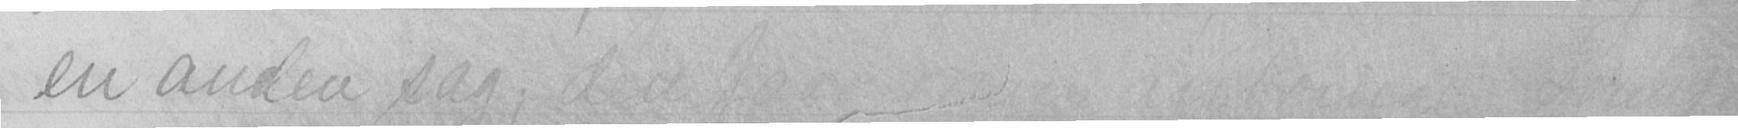en anden sag, den
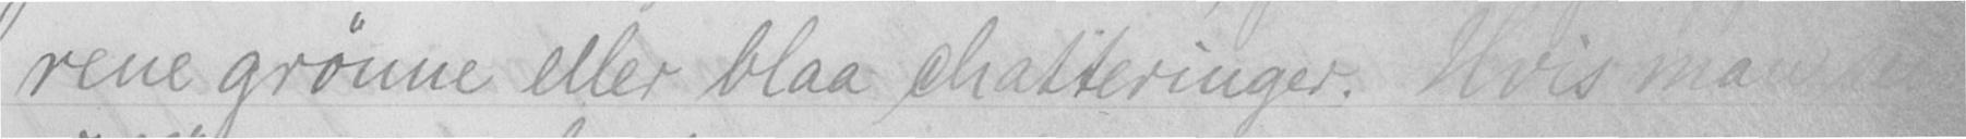rene grønne eller blaa chatteringer. Hvis man
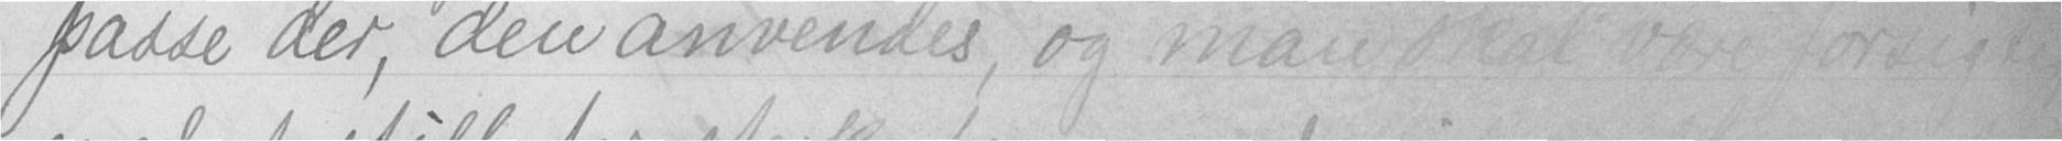passe der, den anvendes, og man skal være forsigtig
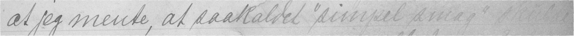at jeg mente, at saakaldet "simpel smag" skulde
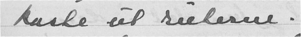kaste ut ruterne.
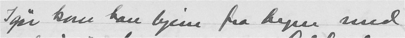Igår kom han hjem fra byen med
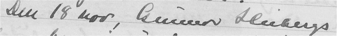Den 18 nov, Gunnar Heibergs
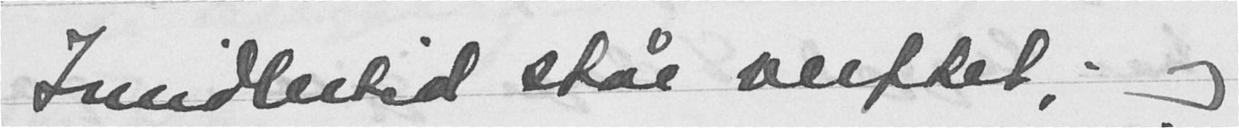Imidlertid står verftet, og
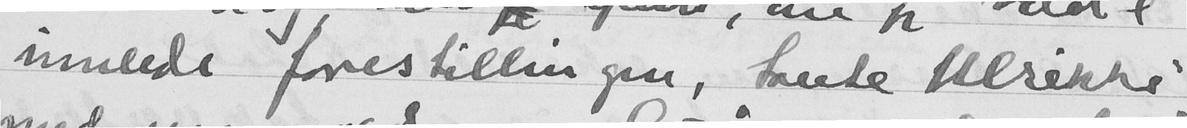innlede forestillingen, "Tante Ulrikke"
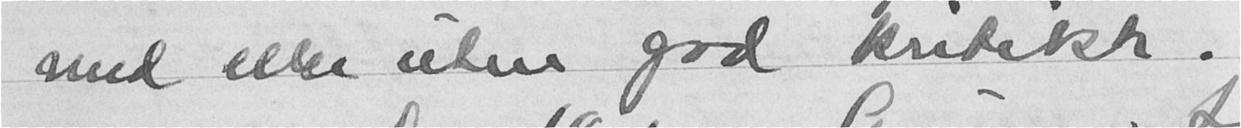med eller uten god kritikk.
